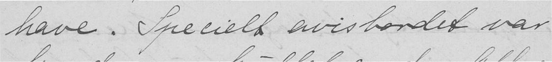have. Specielt avisbordet var
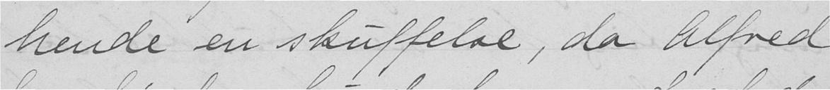hende en skuffelse, da Alfred
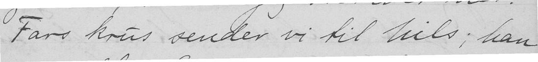Fars krus sender vi til hils; han
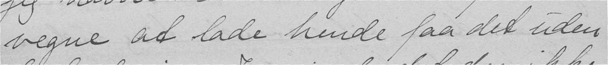vegne at lade hende faa det uden
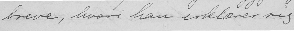breve, hvori han erklærer sig
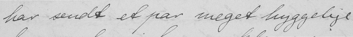har sendt et par meget hyggelige
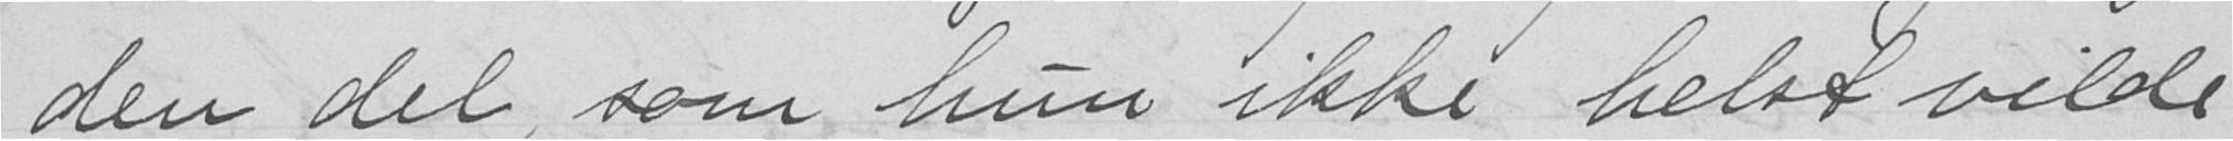den del, som hun ikke helst vilde
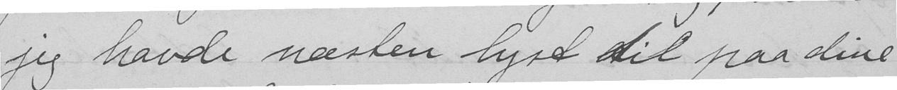jeg havde næsten lyst til paa dine
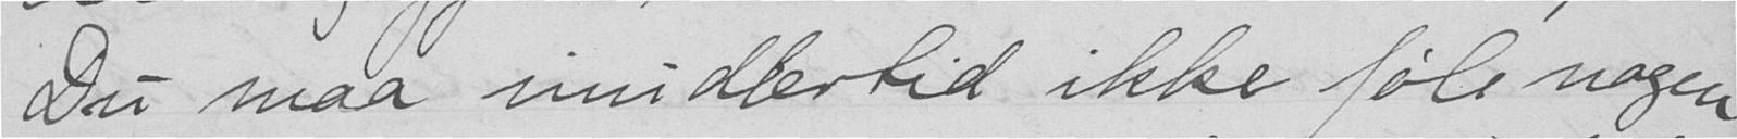Du maa imidlertid ikke føle nogen
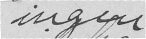ingen
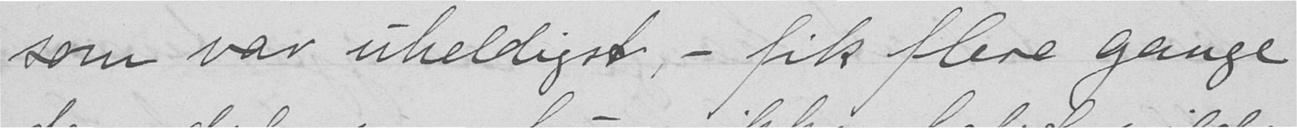som var uheldigst, - fik flere gange
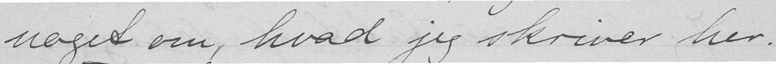noget om, hvad jeg skriver her.
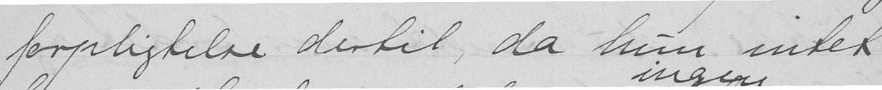forpligtelse dertil, da hun intet
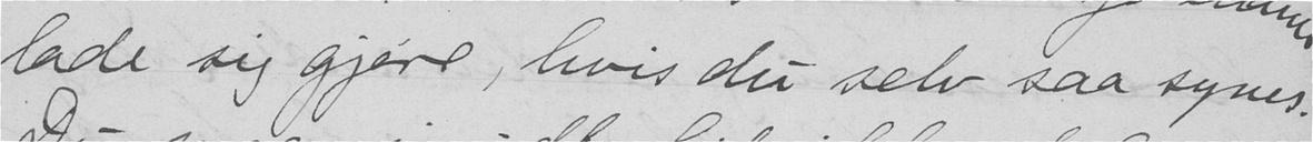lade sig gjøre, hvis du selv saa synes.
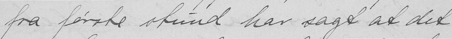fra første stund har sagt at det
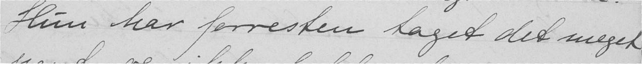Hun har forresten taget det meget
